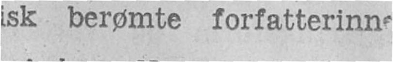isk berømte forfatterinne
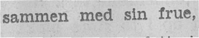sammen med sin frue,
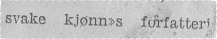svake kjønn"s forfatteri
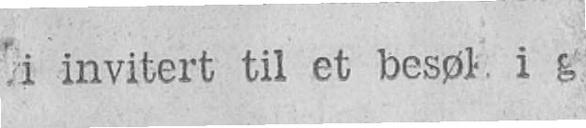i invitert til et besøk i g
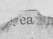ea
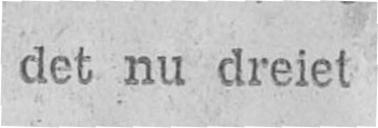det nu dreiet
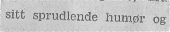sitt sprudlende humor og
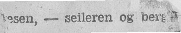esen,— seileren og berg
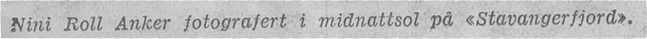Nini Roll Anker fotografert i midnattsol på "Stavangerfjord".
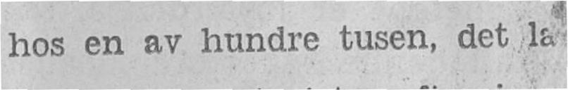hos en av hundre tusen, det la
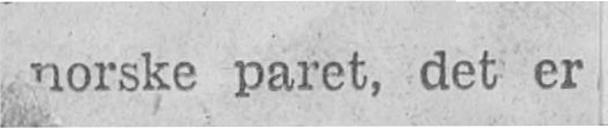norske paret, det er
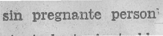sin pregnante person
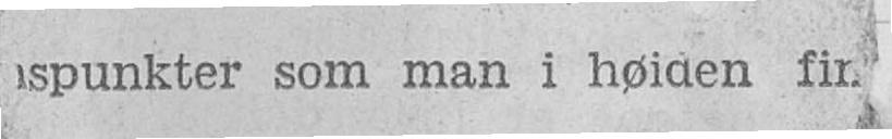ispunkter som man i høiden fir
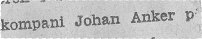kompani Johan Anker p
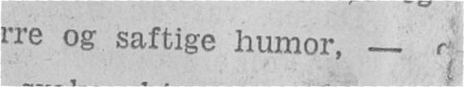ørre og saftige humor, -
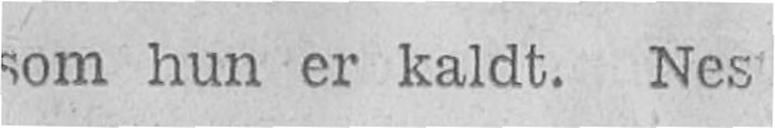som hun er kaldt. Nes
